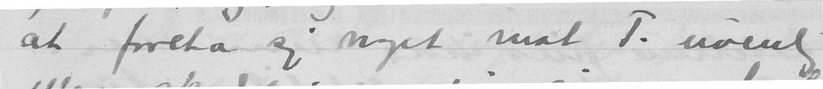at foreta sig noget mot T. uvenlig
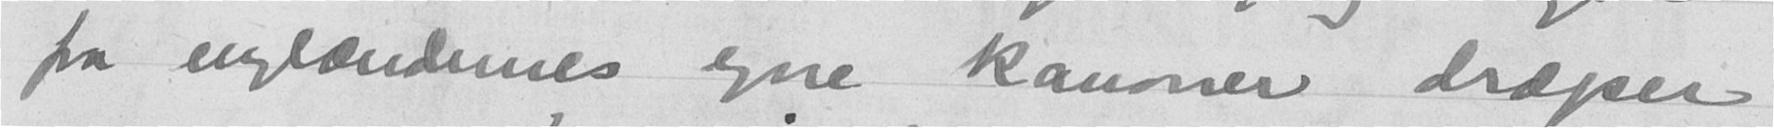fra englændernes egne kanoner dræper
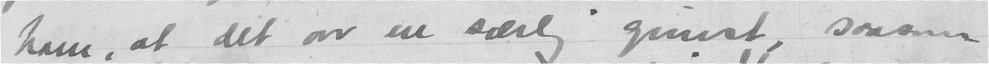ham, at det var en særlig gunst, saasom
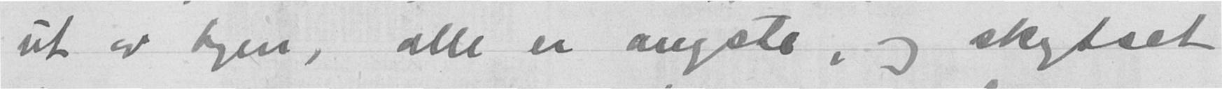ut av byen, alle er angste, og skytset
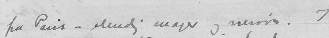fra Paris - elendig mager og nervøs. I
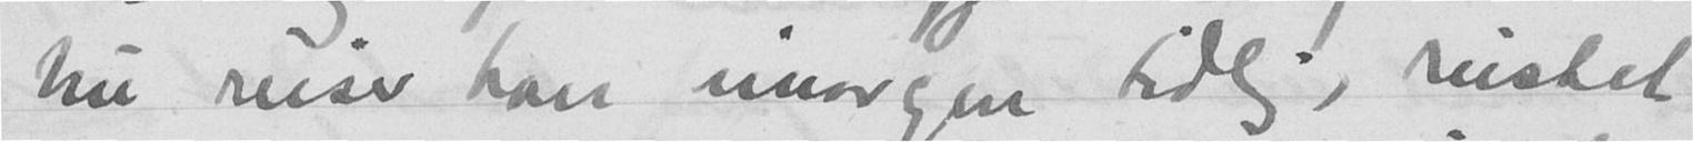Nu reiser han imorgen tidlig, rustet
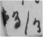3/3
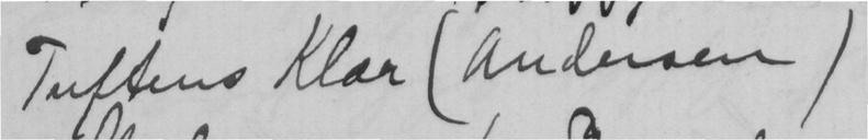Tuftens Klær (Andersen)
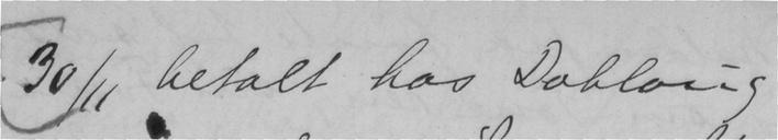30/11 betalt hos Dobloug
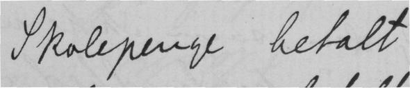Skolepenge betalt
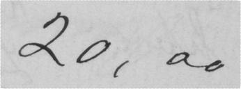20, 00
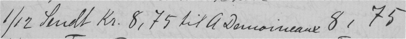1/12 Sendt Kr. 8, 75 til A Demoineaux 8, 75
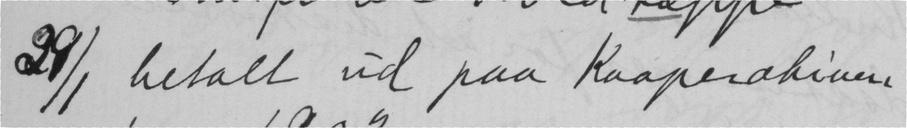29/1 betalt ud paa Kvaperahiven
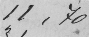11.70
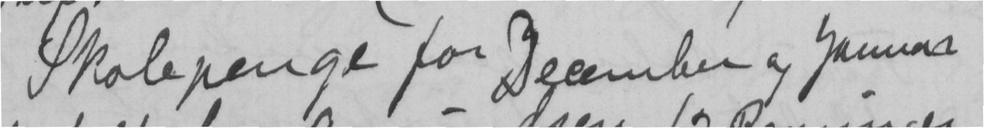Skolepenge for December og januar
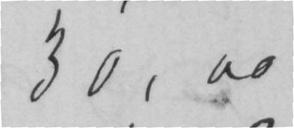30, 00
